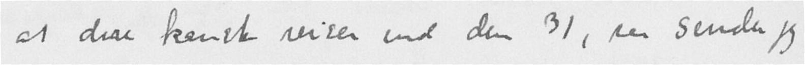at dere kanske reiser ind den 31, saa sender jeg
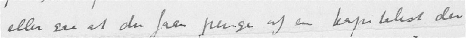eller saa at du faar penger af en kapitalist der
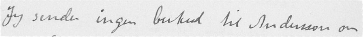Jeg sender ingen besked til Andersson om
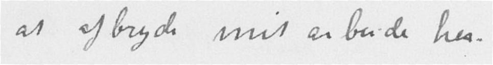at afbryde mit arbeide her.
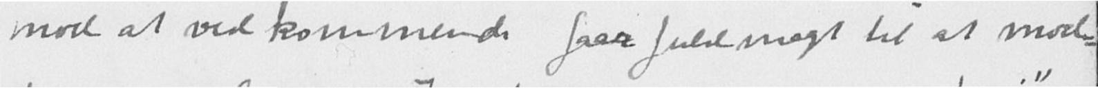mod at vedkommende faar fuldmagt til at mod-
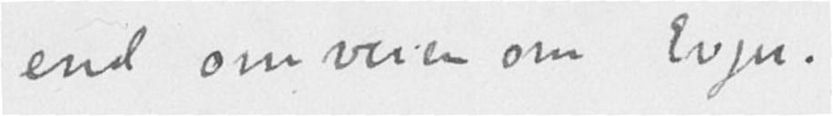end omveien om Evju.
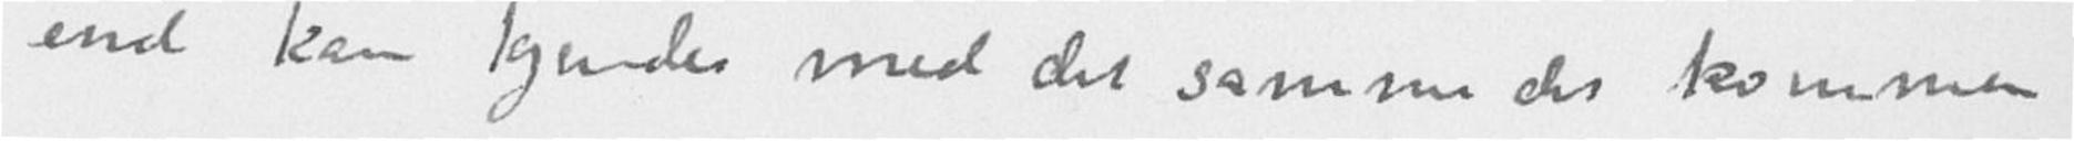end kan kjendes med det samme det kommer
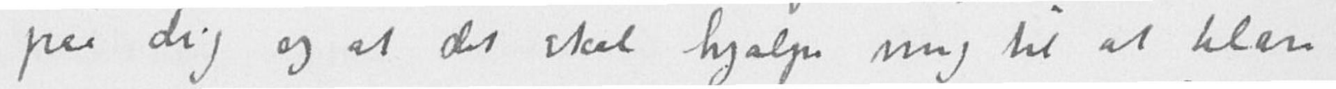paa dig og at det skal hjælpe mig til at klare
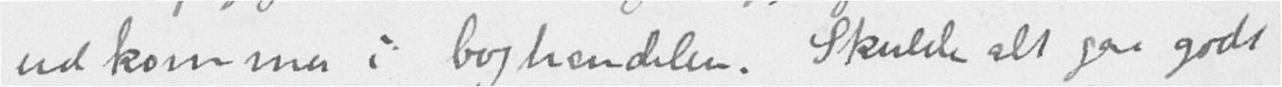udkommer i boghandelen. Skulde alt gaa godt
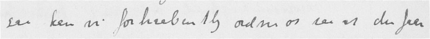saa kan vi forhaabentlig ordne os saa at du faar
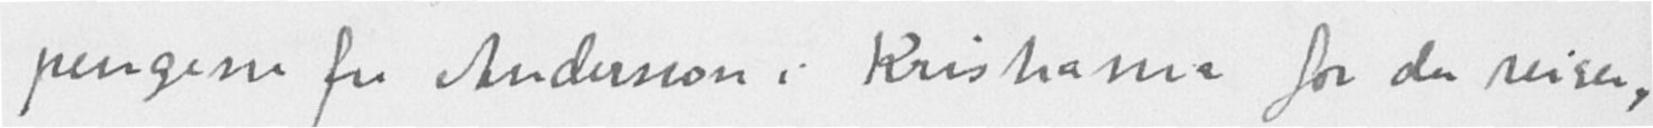pengene fra Andersson i Kristiania før du reiser,
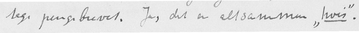tage pengebrevet. Ja, det er altsammen "hvis".
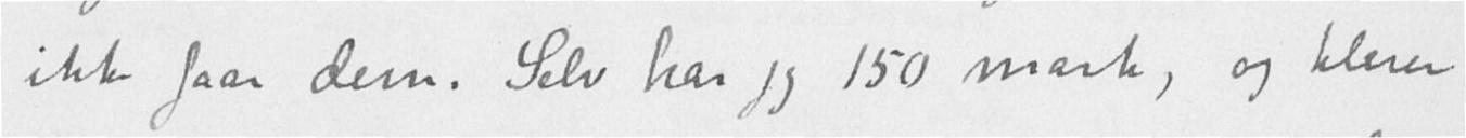ikke faar dem. Selv har jeg 150 mark, og klarer
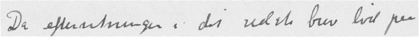Da efterretninger i det sidste brev lød paa
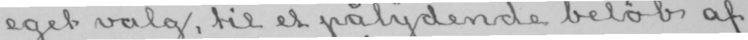eget valg, til et pålydende beløb af
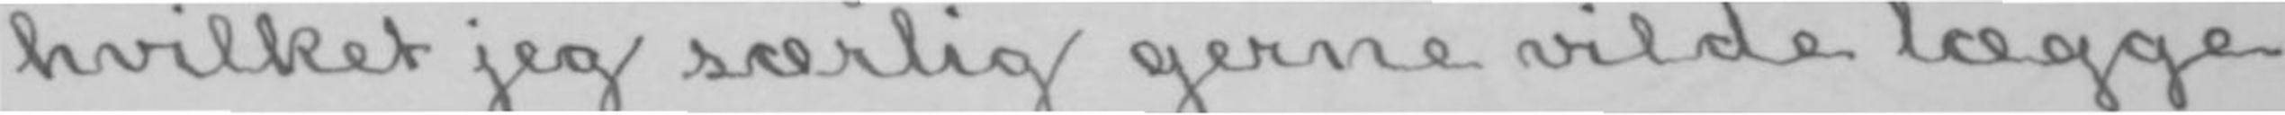hvilket jeg særlig gerne vilde lægge
